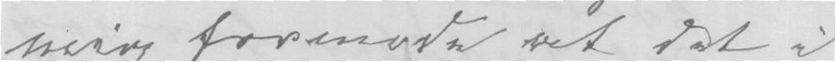mig formode at det i
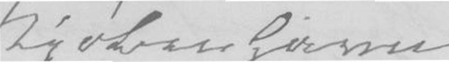Kjøbenhavn
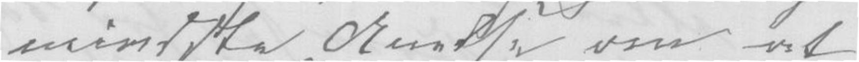mindste Anelse om at
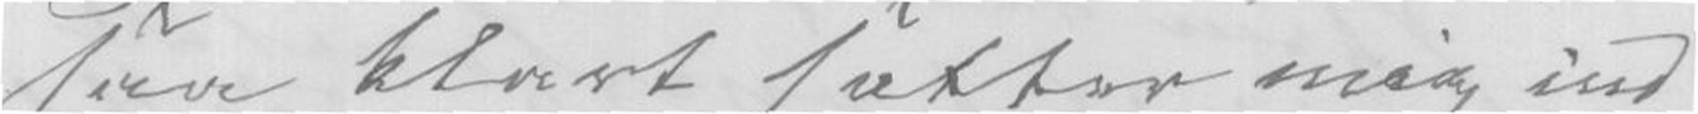saa klart setter mig ind
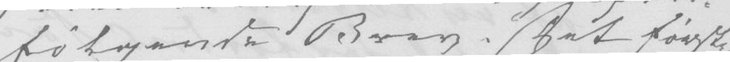følgende Brev. Det første
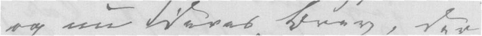og nu Deres Brev, der
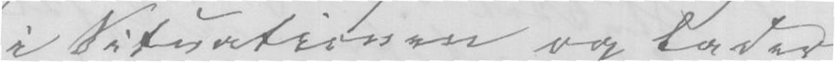i Situationen og lader
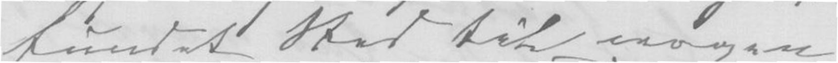fundet Sted til nogen
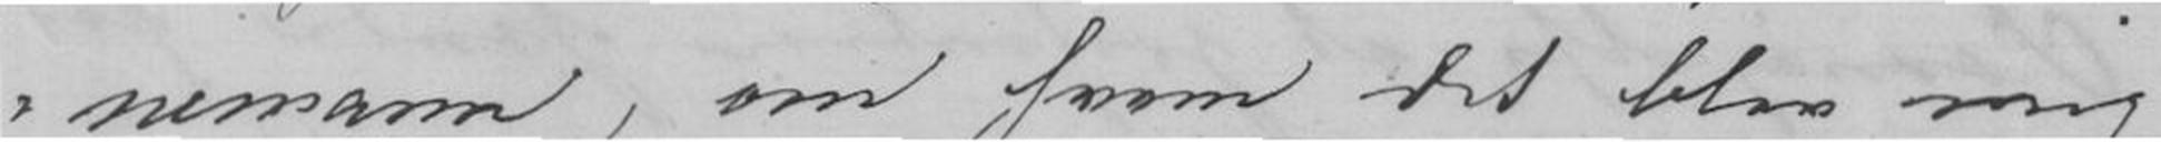nemann, om hvem det blev mig
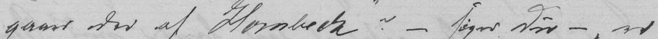gaar der af Hornbeck - siger du -, er
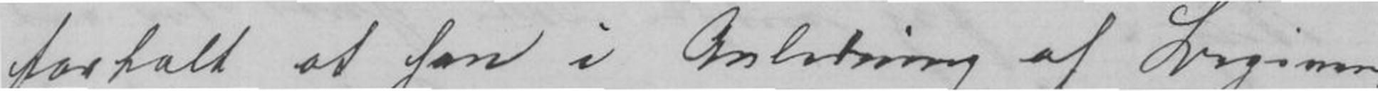fortalt at han i Anledning af Begiven
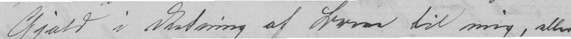Gjæld i Retning af brev til mig, eller
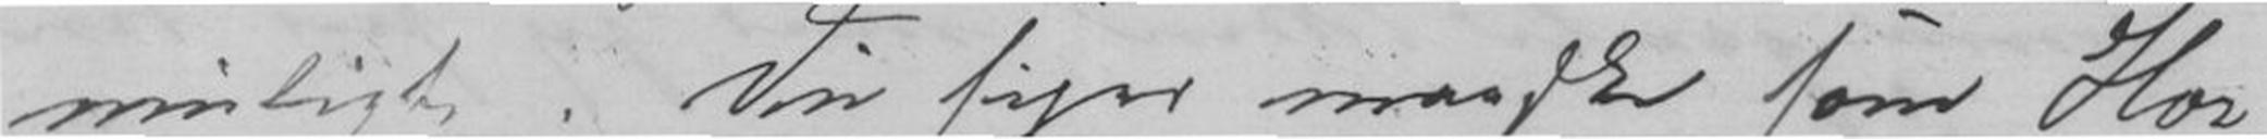muligt. Du siger maaske som Hor
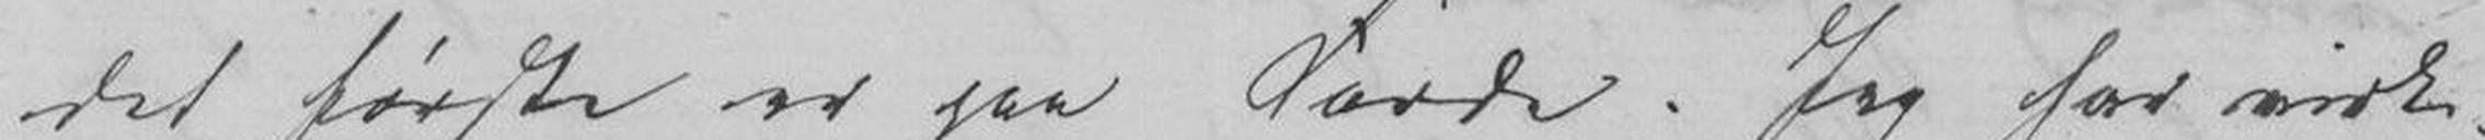det første er paa færde. Jeg har virke
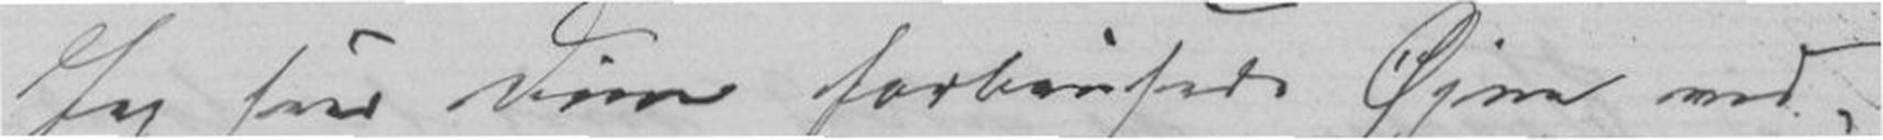Jeg ser dine forbausede Øjne mod
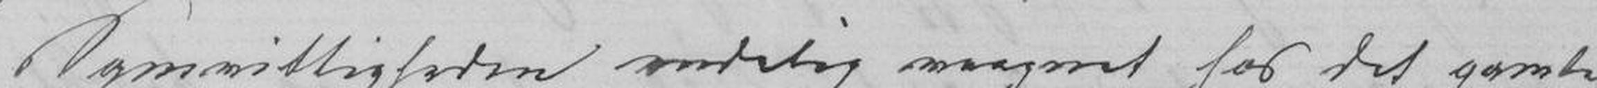samvittigheden endelig vaagnet hos det gamle
